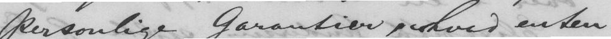personlige Garantier, hvad enten
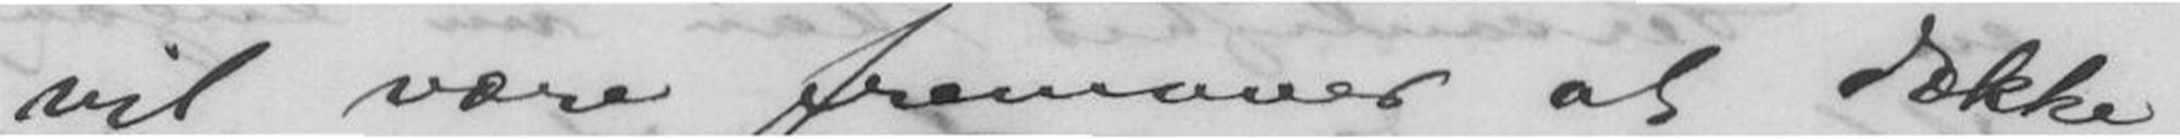vil være fremover at dække
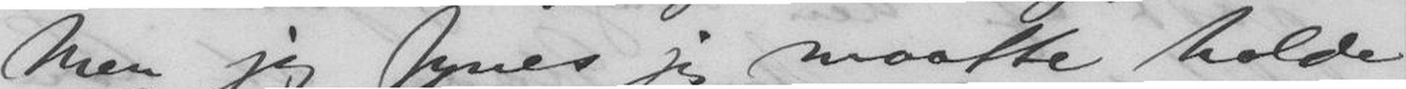Men jeg synes jeg maatte holde
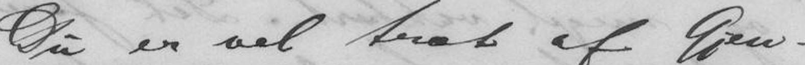Du er vel træt af Gjen-
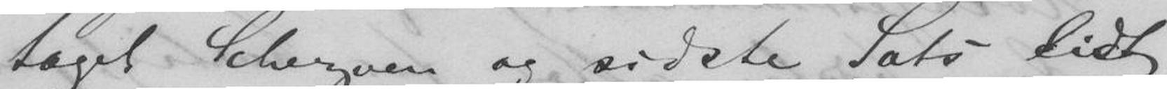taget Scherzoen og sidste Sats lidt
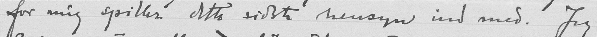for mig spiller dette sidste hensyn ind med. Jeg
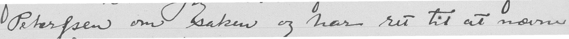Peterssen om saken og har ret til at nævne
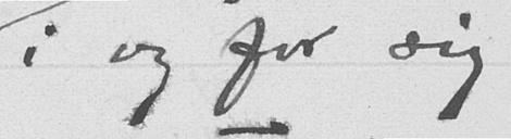i og for sig
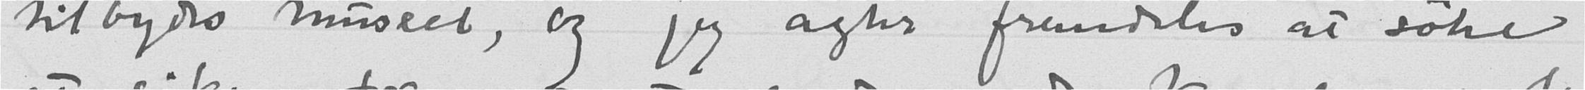tilbydes museet, og jeg agter fremdeles at søke
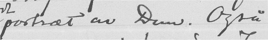portræt av Dem. Også
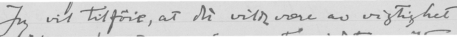Jeg vil tilføie, at det vilde være av vigtighet
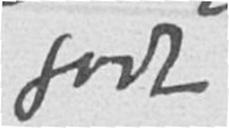godt
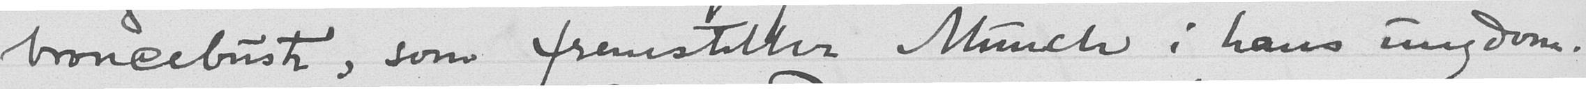broncebuste, som fremsteller Munch i hans ungdom.
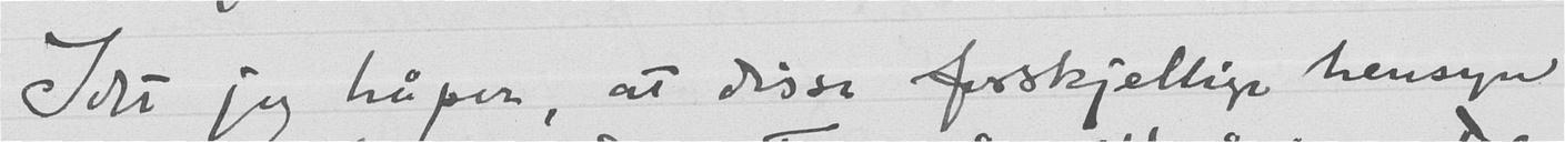Idet jeg håper, at disse forskjellige hensyn
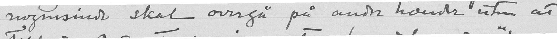nogensinde skal overgå på andre hænder uten at
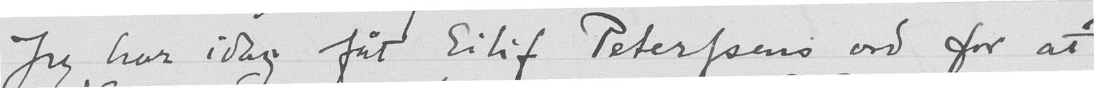Jeg har idag fåt Eilif Peterssens ord for at
